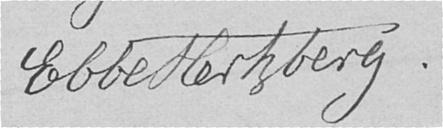Ebbe Hertzberg.
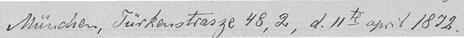München, Türkenstrasse 48, 2, d. 11te april 1872.
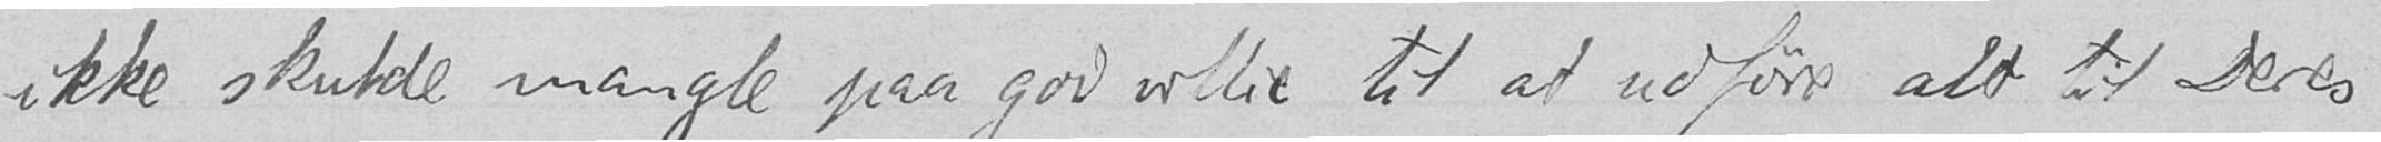ikke skulde mangle paa god villie til at udføre alt til Deres
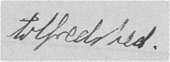tilfredshed.
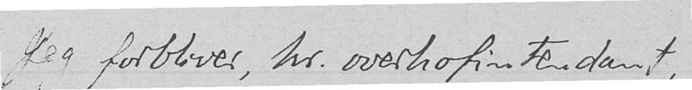Jeg forbliver, hr. overhofintendant,
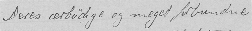Deres ærbødige og meget forbundne
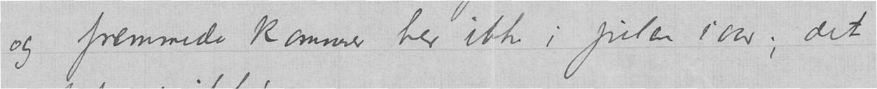og fremmede kommer her ikke i julen iaar; det
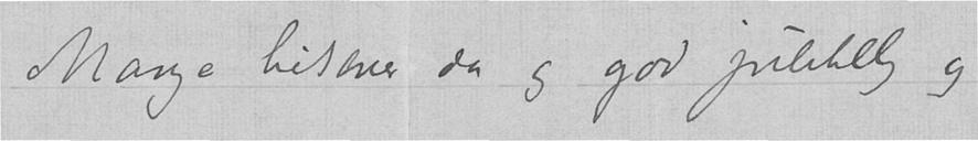Mange hilsener da og god julehelg og
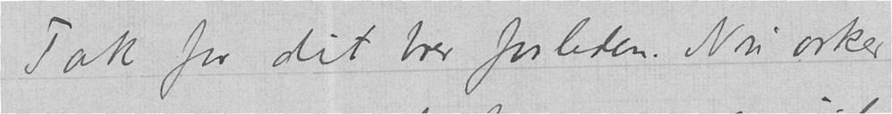Tak for dit brev forleden. Nu orker
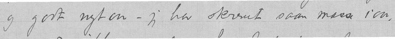og godt nytaar – jeg har skrevet saan masse iaar,
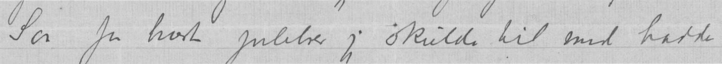Saa for hvert julebrev jeg skulde til med hadde
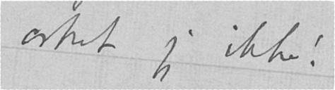orket jeg ikke!
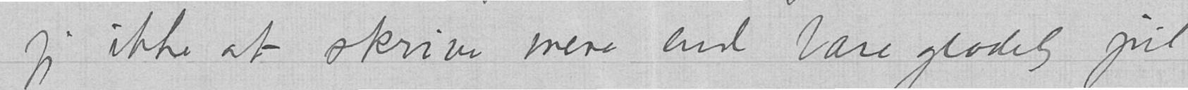jeg ikke at skrive mere end bare glædelig jul
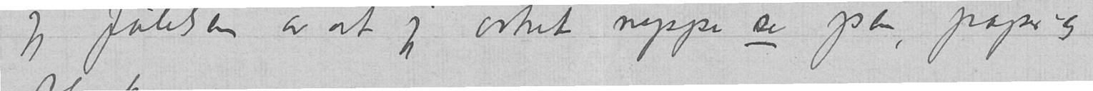jeg følelsen av at jeg orket neppe se pen, papir og
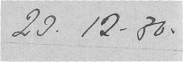23.12. 30.
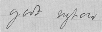godt nytaar
